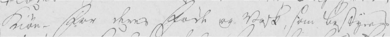Kiøn - For deres Føde og Vask, som bestyres
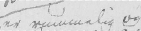er rummelig og
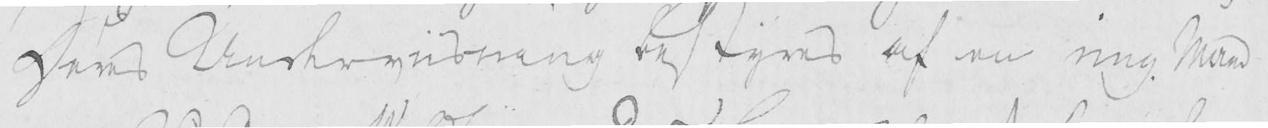Deres Undervisning bestyres af en ung Mand
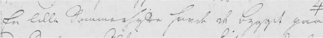En lille Tømmerhytte havde de bygget paa
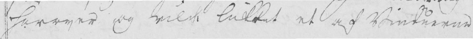herved og vilde lukket et af Vinduerne
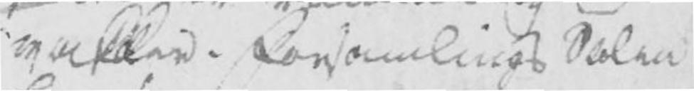vakker. Forsamlings Salen
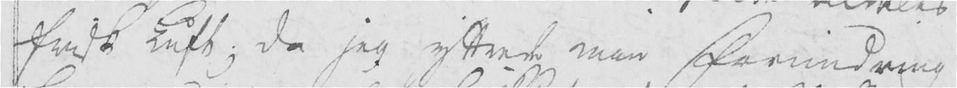frisk Luft; da jeg yttrede min Forundring
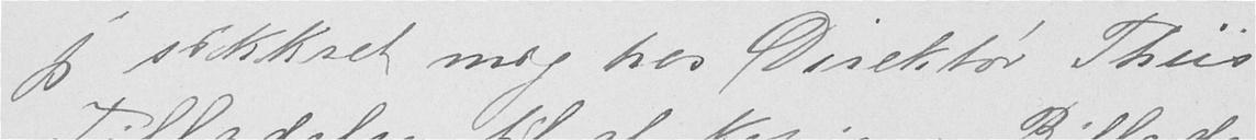jeg sikkret mig hos Direktør Thiis
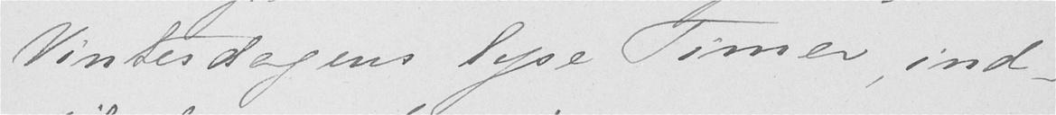Vinterdagens lyse Timer, ind-
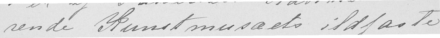rende Kunstmusæets ildfaste
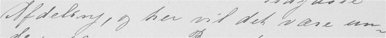Afdeling, og her vil det være un-
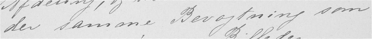der samme Bevogtning som
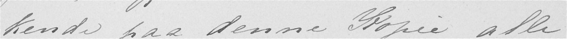kende paa denne Kopie alle
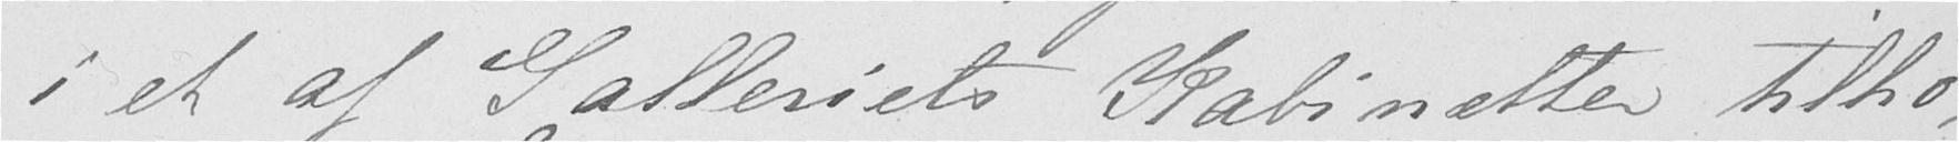i et af Galleriets Kabinetter tilhø-
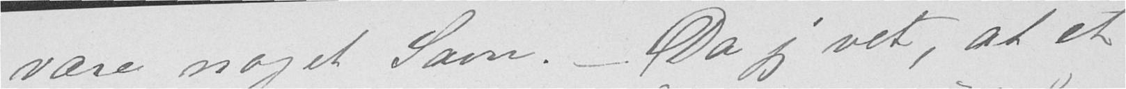være noget Savn. - Da jeg vet, at et
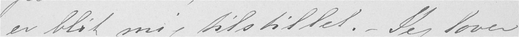er blit mig tilstillet. - Jeg lover
